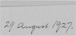29 August 1927.
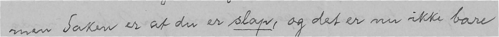men Saken er at du er slap, og det er nu ikke bare
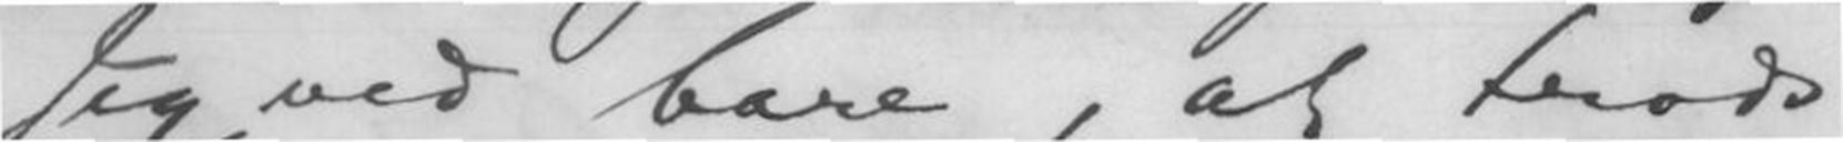Jeg ved bare, at trods
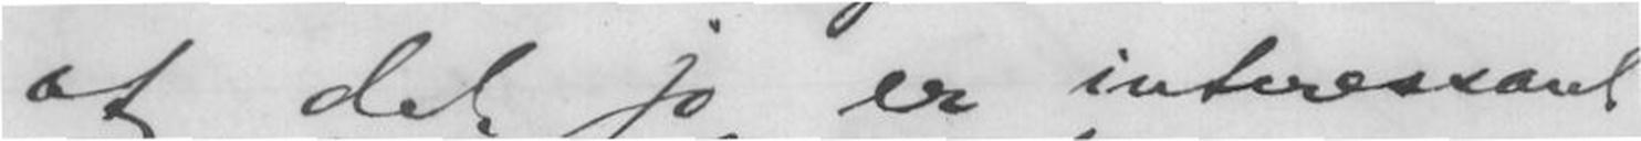at det jo er interessant
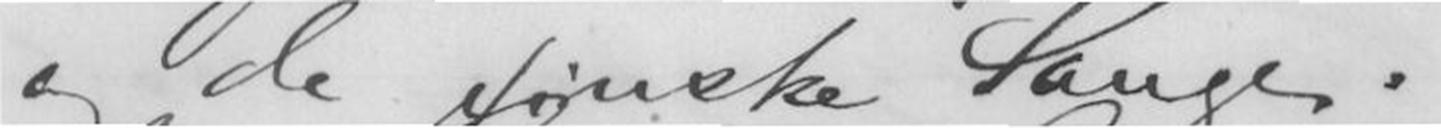og de finske Sange.
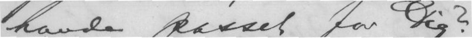havde passet for Dig?
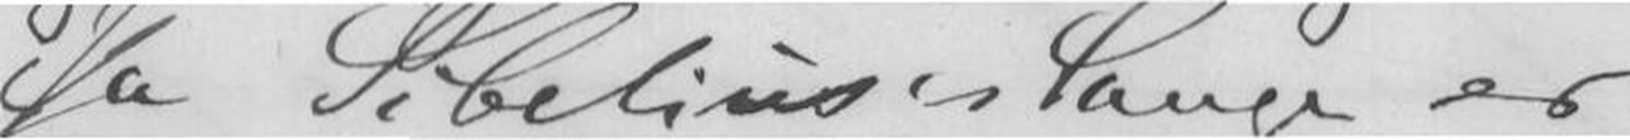Ja Sibelius's Sange er
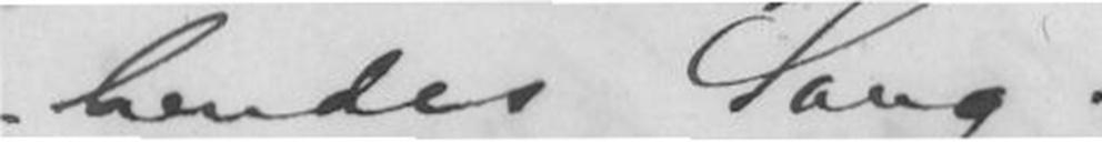efter hendes Sang.
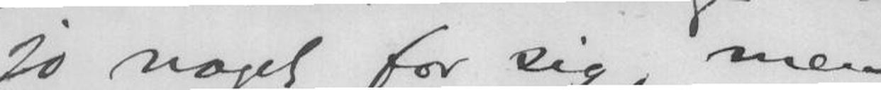jo noget for sig, men
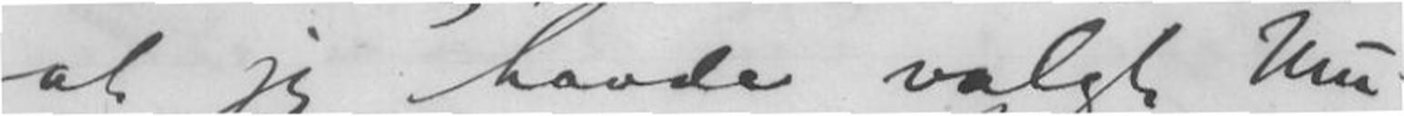- at jeg havde valgt Mu-
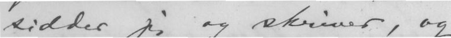sidder jeg og skriver, og
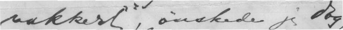vakkert", ønskede jeg dog,
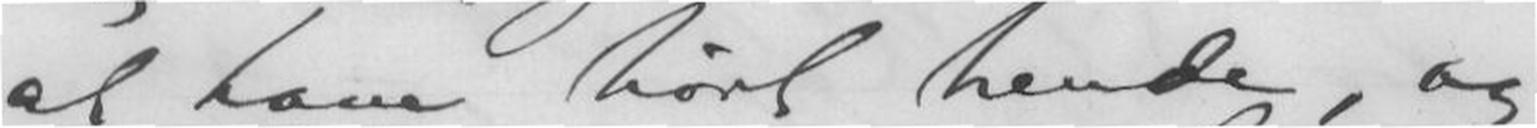at have hørt hende, og
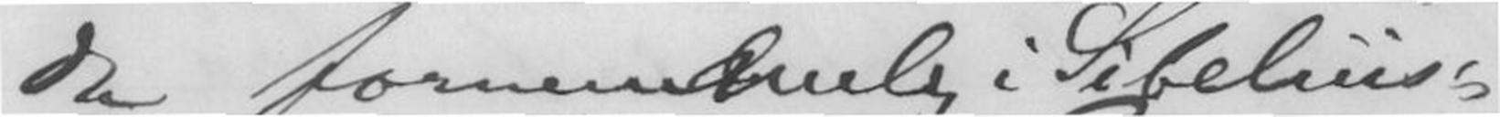da fornemmelig i Sibelius's
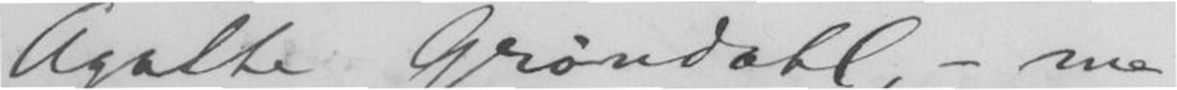Agathe Grøndahl, - me-
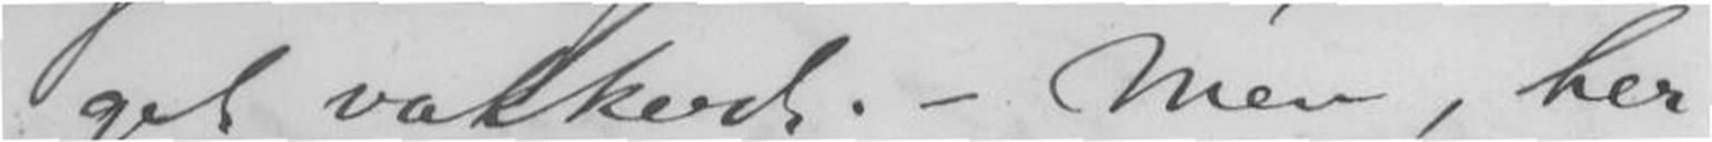get vakkert. - Men, her
